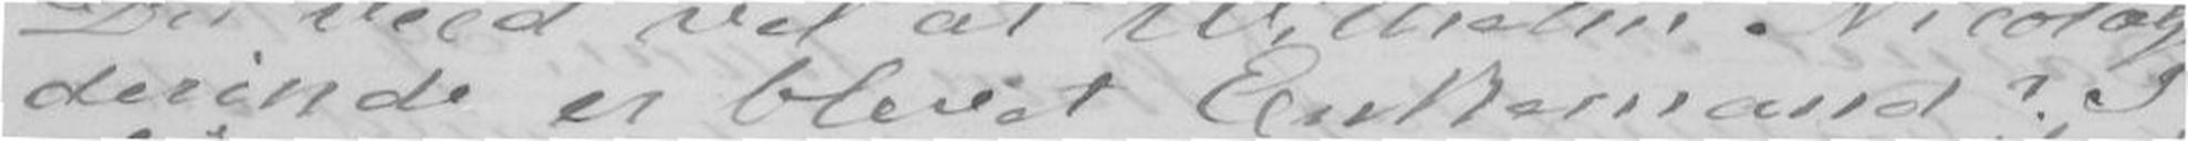derinde er blevet Enkemand? I
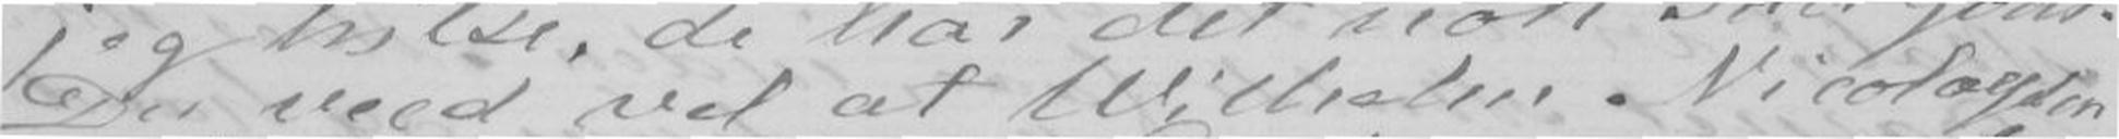Du veed vel at Wilhelm Nicolaysen
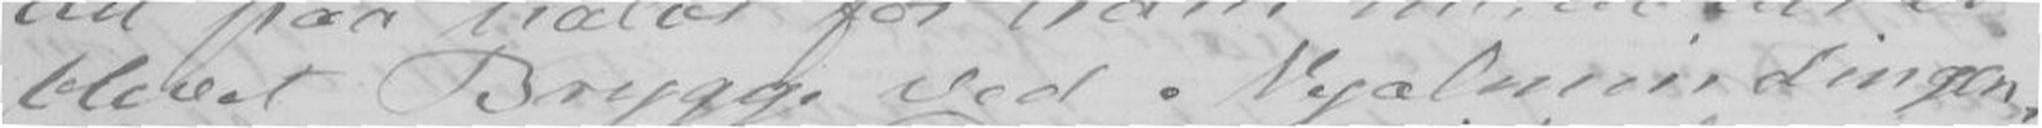blevet Brygge ved Nyalmendingen,
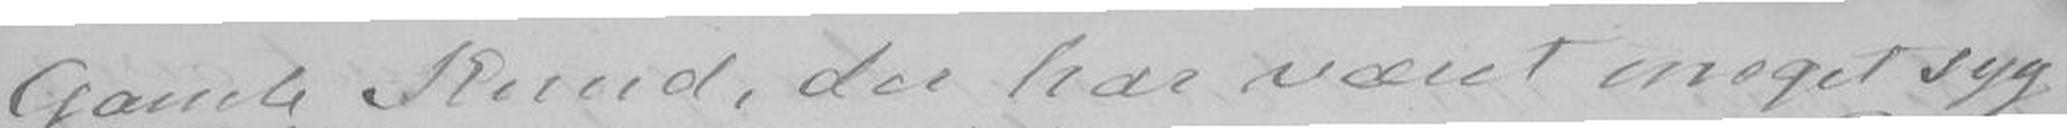Gamle Knud, der har været meget syg
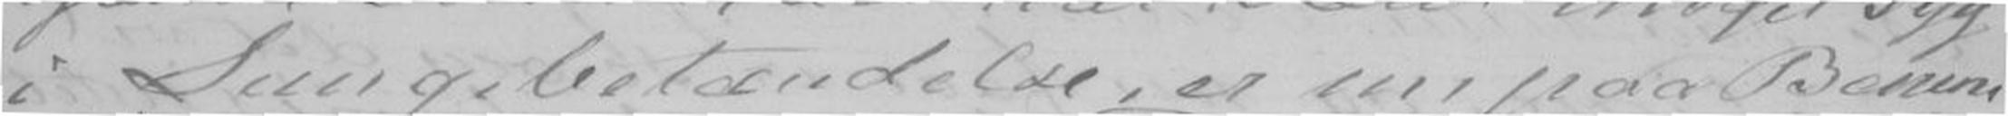i Lungebetændelse, er nu paa Benene
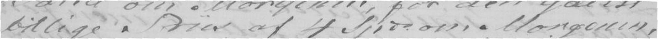billige Pris af 4 Spd om Morgenen,
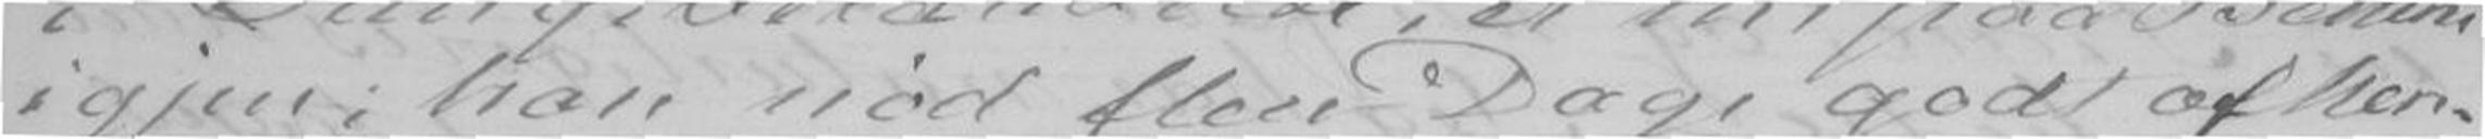igjen; han nød flere Dage godt af hen-
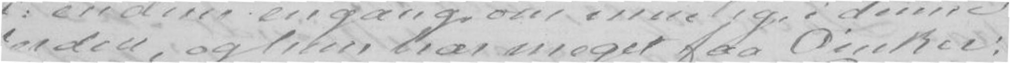den, og hun har meget faa Ønsker;
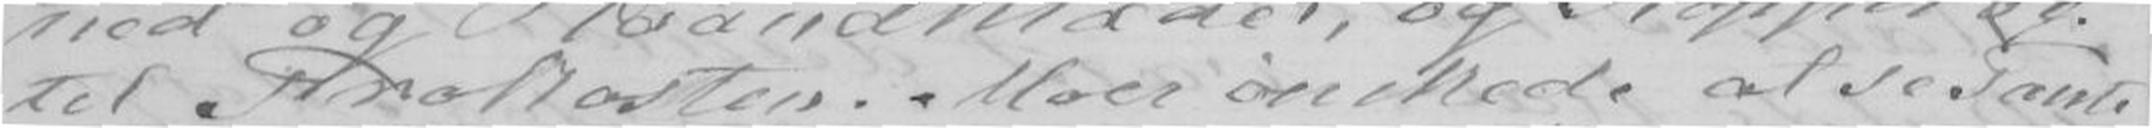til Frokosten. Moer ønskede at se Tante
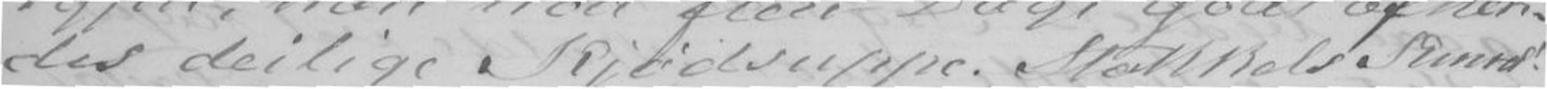des deilige Kjødsuppe. Stakkels Knud!
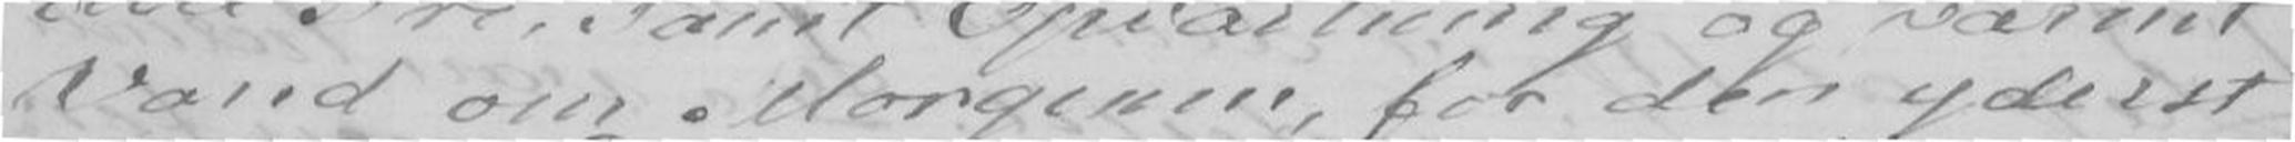Vand om Morgenen, for den yderst
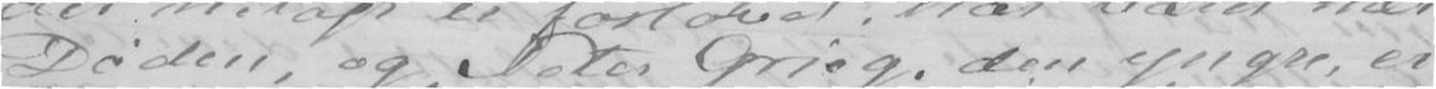Døden, og Peter Grieg, den yngre, er
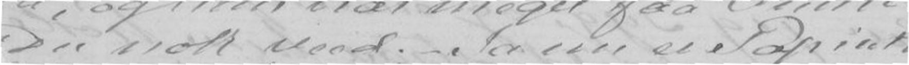Du nok veed. - Ja nu er Papiret
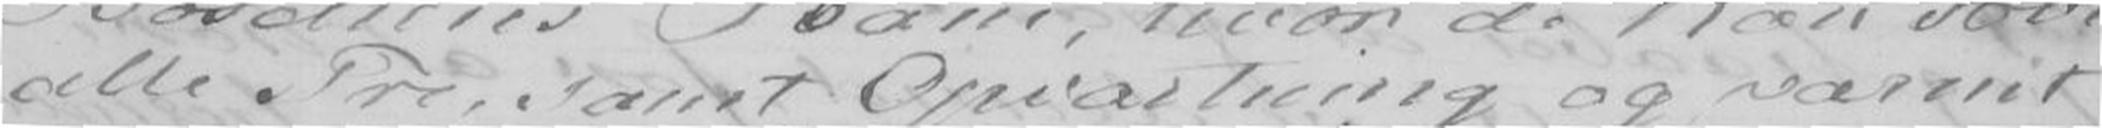alle Tre, samt Opvartning og varmt
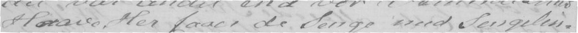Haave. Her faaer de Senge med Sengelin-
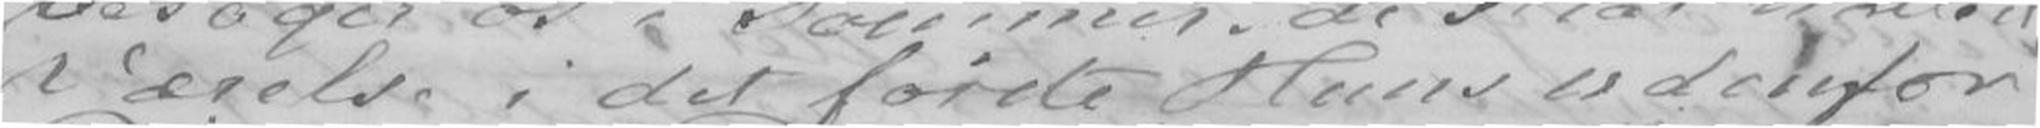Værelse i det første Huus udenfor
